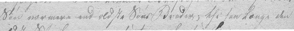Søn nærmere end ældste Søns Broder; thi saa længe den
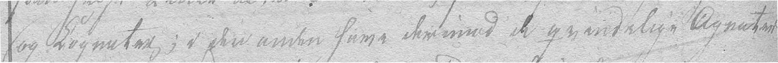og Cognater; i den anden have derimod de qvindelige Agnater
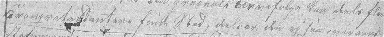Kronpretendentere finde Sted, deels er den ej saa overeens-
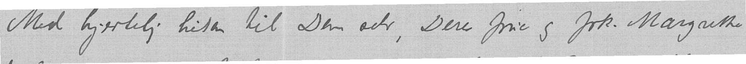Med hjertelig hilsen til Dem selv, Deres frue og frk. Margrethe
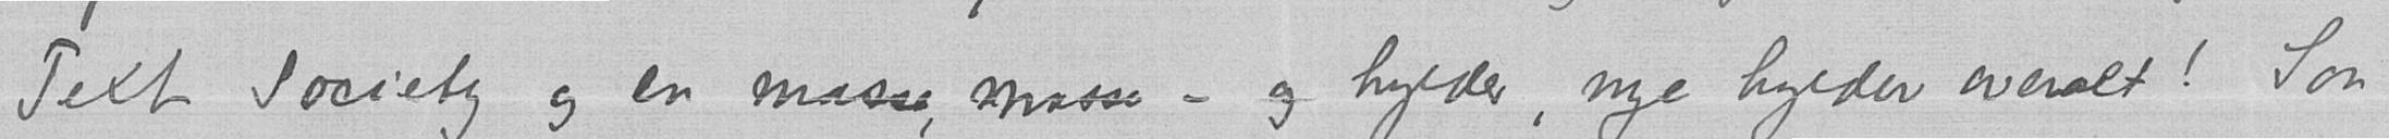Text Society og en masse, masse – og hylder, nye hylder overalt! Saa
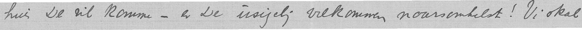hvis De vil komme – er De usigelig velkommen naarsomhelst! Vi skal
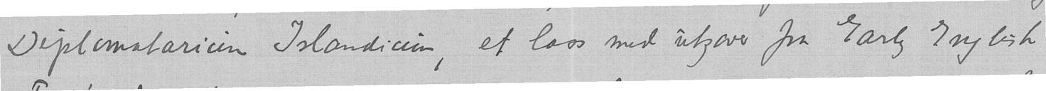Diplomatarium Islandicum, et kaos med utgaver fra Early English
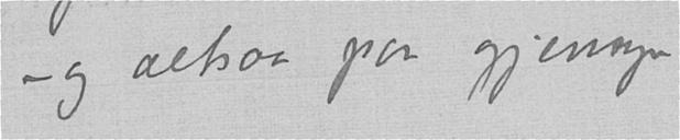Deres hengivne
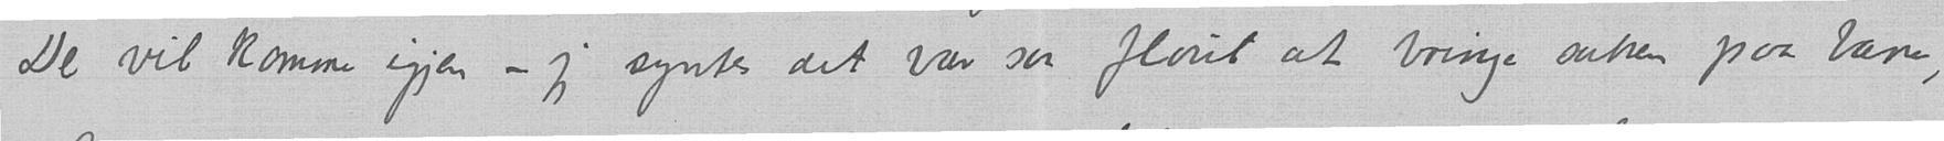De vil komme igjen – jeg syntes det var saa flaut at bringe saken paa bane
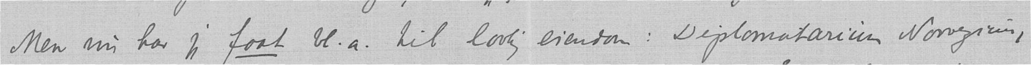Men nu har jeg faat bl.a. til lovlig eiendom: Diplomatarium Norvegicum,
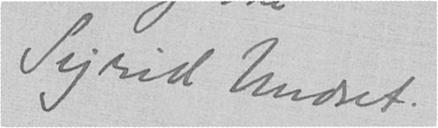Sigrid Undset.
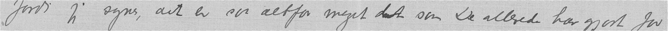fordi jeg synes, det er saa altfor meget det som De allerede har gjort for
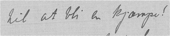til at bli en kjæmpe!
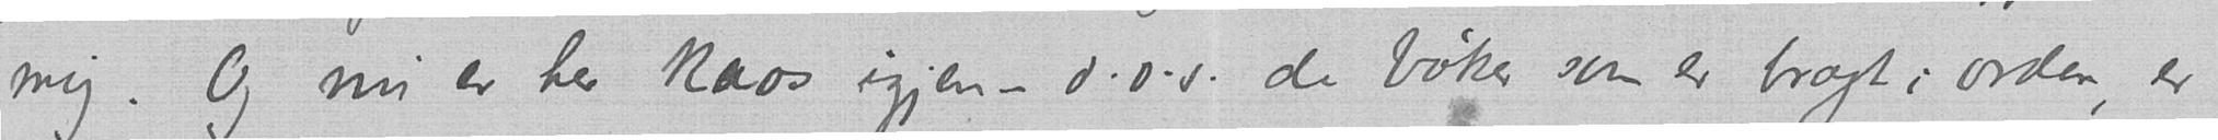mig. Og nu er her kaos igjen – d.v.s. de bøker som er bragt i orden, er
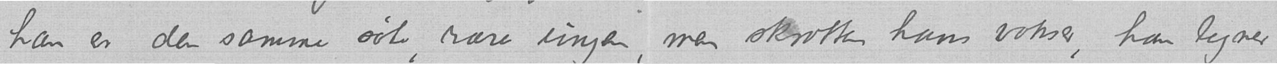han er den samme søte, rare ungen, men skrotten hans vokser, han tegner
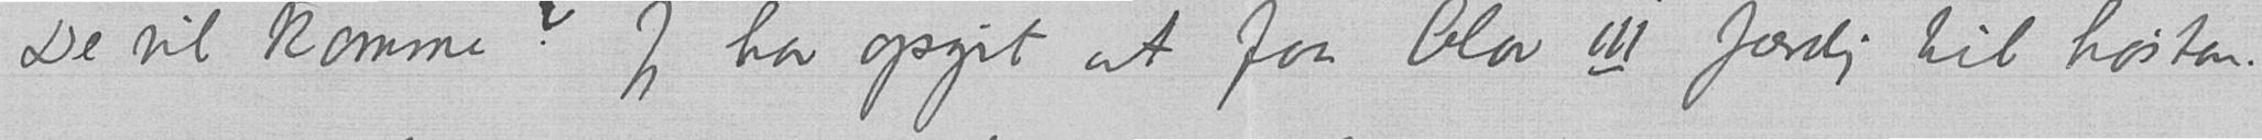De vil komme? Jeg har opgit at faa Olav III færdig til høsten.
